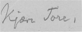Kjære Tore,
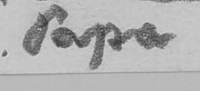Papa
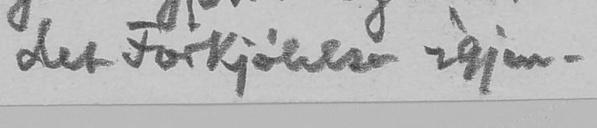det Forkjølelse igjen.
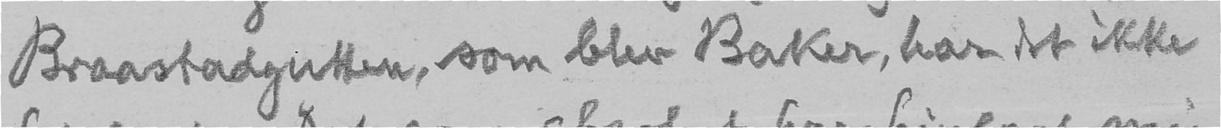Braastadgutten, som blev Baker, har det ikke
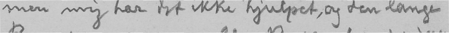men mig har det ikke hjulpet, og den lange
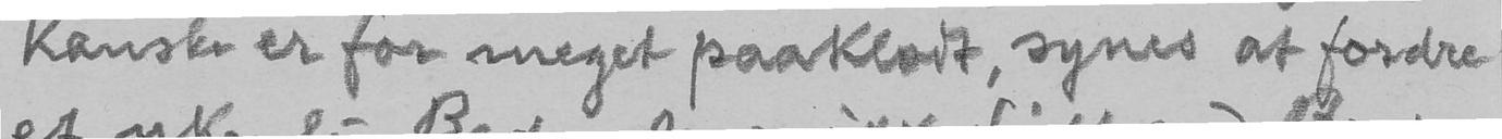kanske er for meget paaklædt, synes at fordre
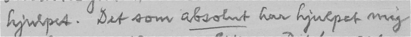hjulpet. Det som absolut har hjulpet mig
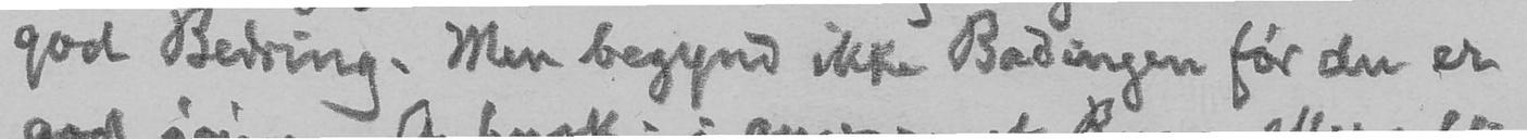god Bedring. Men begynd ikke Badingen før du er
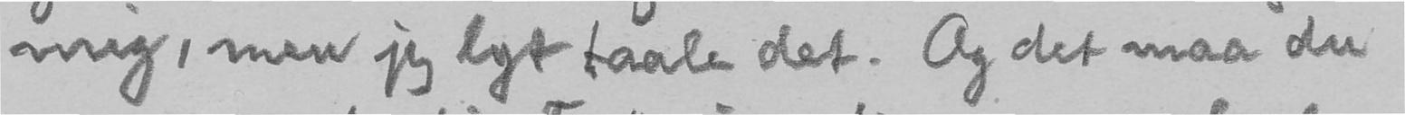mig, men jeg lyt taale det. Og det maa du
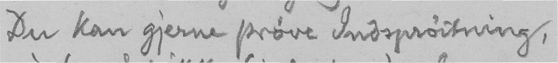Du kan gjerne prøve Indsprøitning,
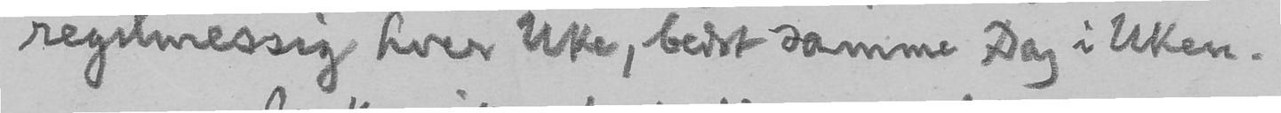regelmessig hver Uke, bedst samme Dag i Uken.
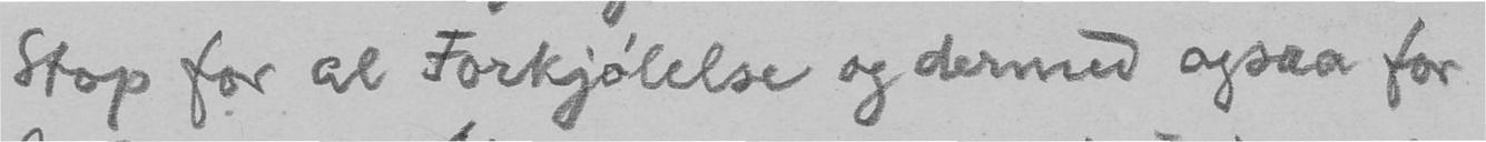Stop for al Forkjølelse og dermed ogsaa for
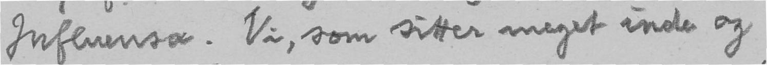Influensa. Vi, som sitter meget inde og
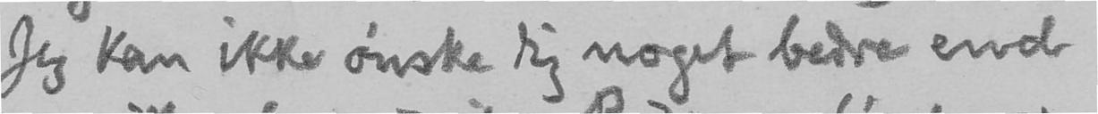Jeg kan ikke ønske dig noget bedre end
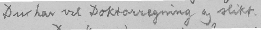Du har vel Doktorregning og slikt.
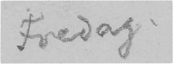Fredag.
